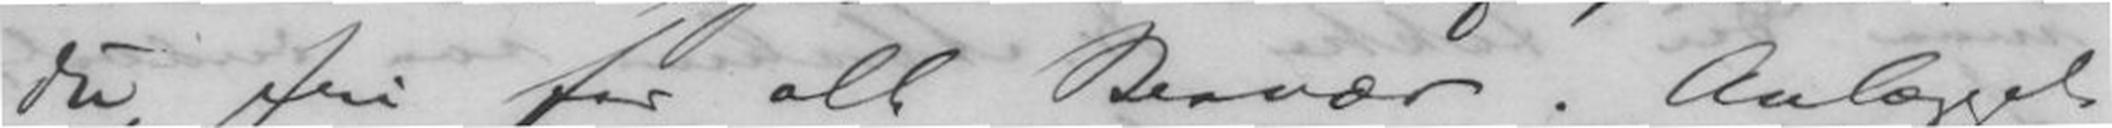du fri for alt Besvær. Anlægget
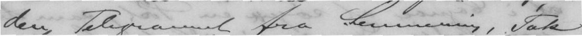den Telegrammet fra Semmering, Tak
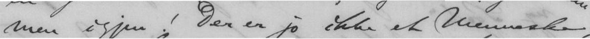men igjen! Der er jo ikke et Menneske,
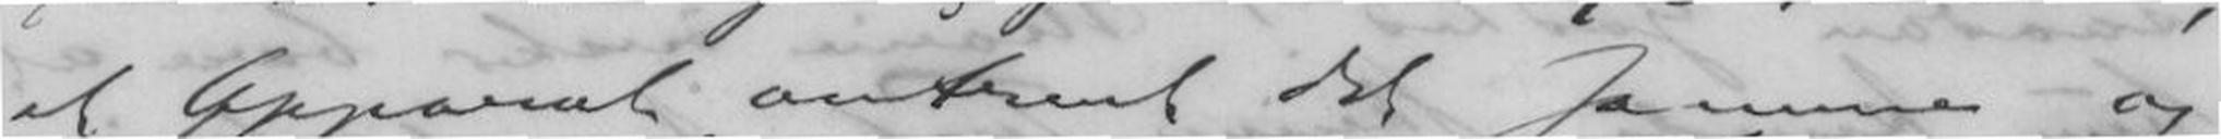et Apparat omtrent det samme og
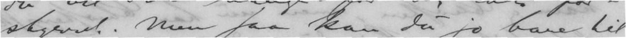styrret. Men saa kan du jo bare til
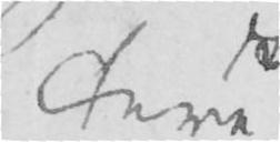tere
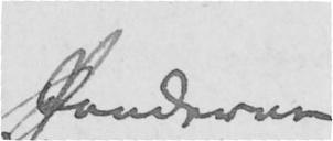Fanderne
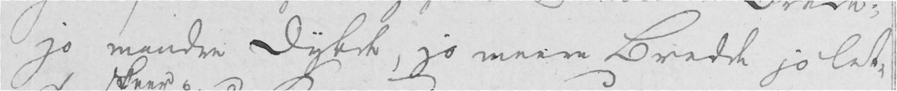jo mindre Dybde, jo meere Bredde jo let-
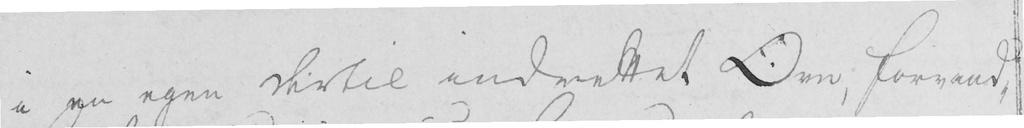i en egen dertil indrettet Ovn, forvand-
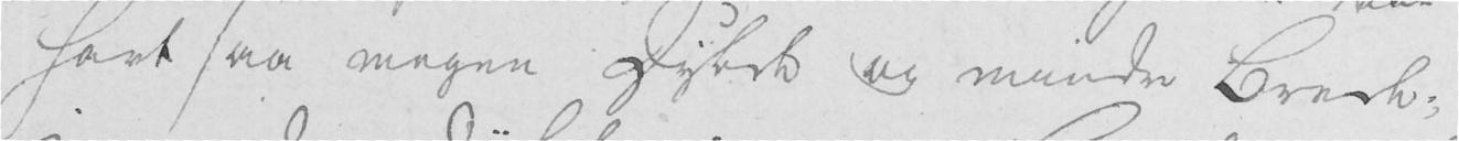havt saa megen Dybde og mindre Brede;
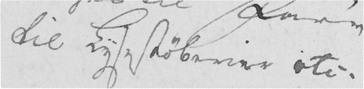til Lysestøberier etc.
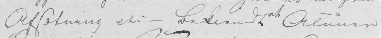Afsætning etc - Bekiendt at Alunen
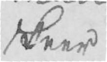skeer
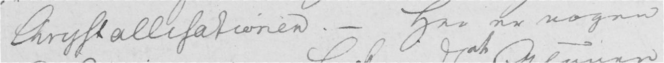Chrystallisationen. - Her er nogen
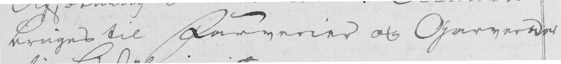bruges til Farverier og Garveret
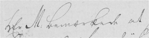Hr M. bemærkede at
